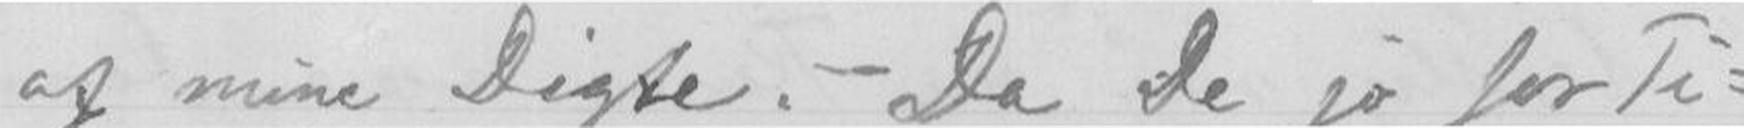af mine Digte. - Da De jo for Ti-
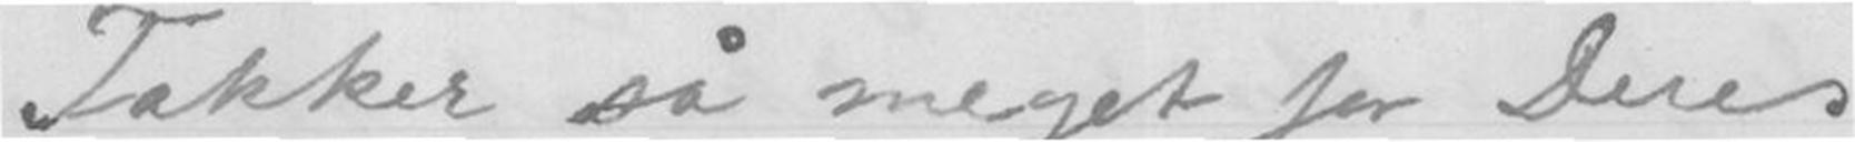Takker så meget for Deres
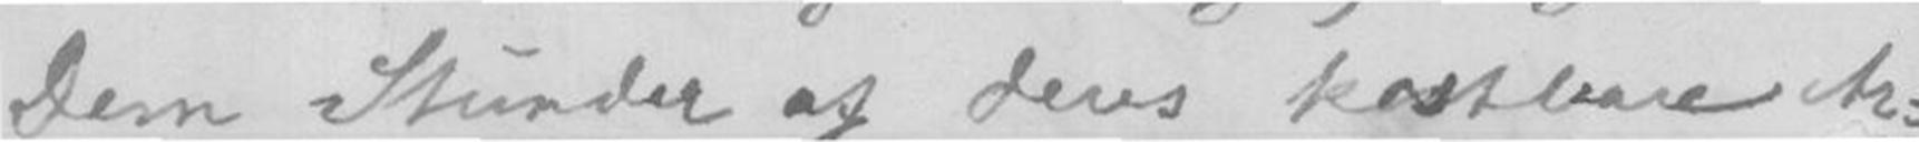Dem Stunder af Deres kostbare Ar-
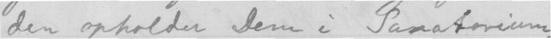den opholder Dem i Sanatorium,
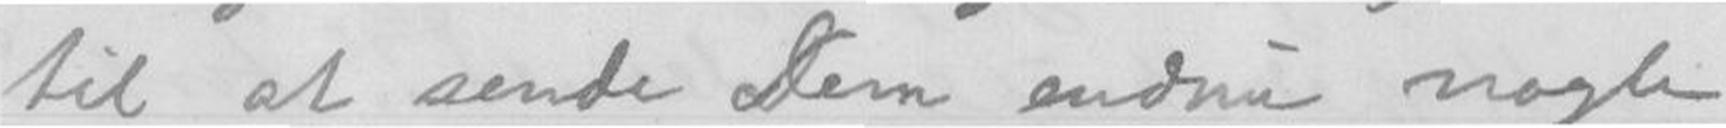til at sende Dem endnu nogle
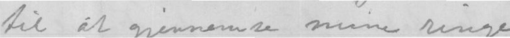til at gjennemse mine ringe
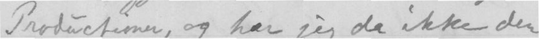Productioner, og har jeg da ikke den
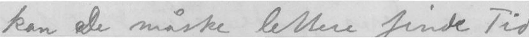kan De måske lettere finde Tid
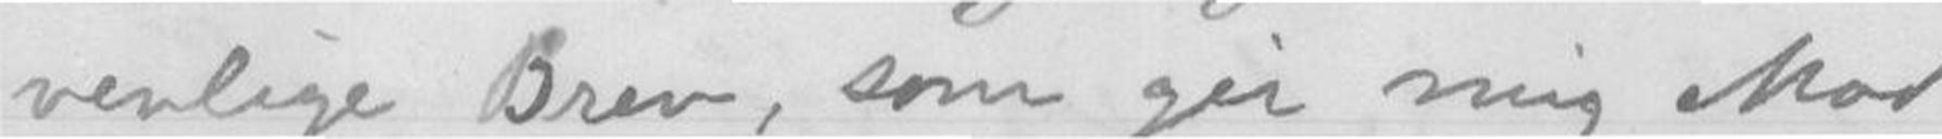venlige Brev, som gir mig Mod
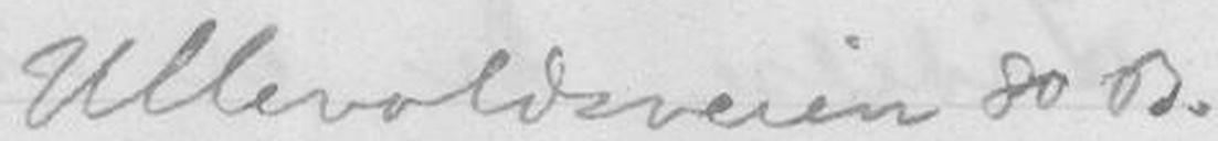Ullevoldsveien 80 B.
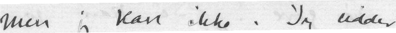Men jeg kan ikke. Jeg sidder
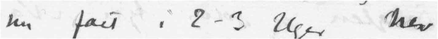nu fast i 3-4 Uger her
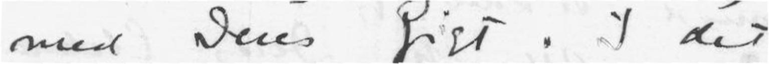med Deres Gigt. I det
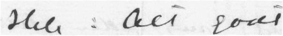Hele: Alt godt!
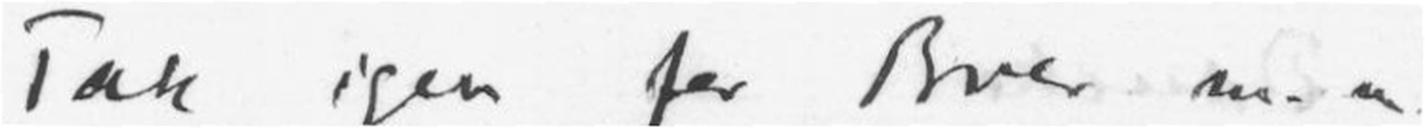og Tak igen for Brev m. m.
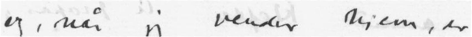og, når jeg vender hjem, er
